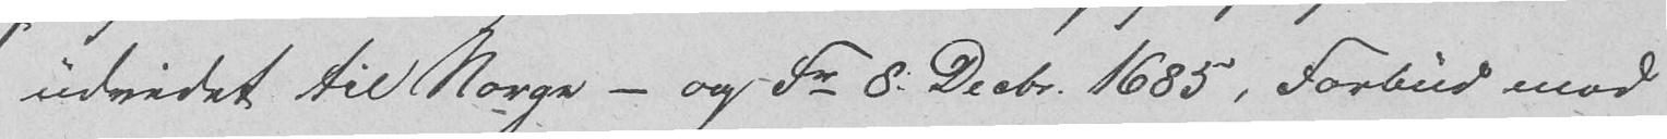udvidet til Norge - og Fr 8. Decbr. 1685, Forbud mod
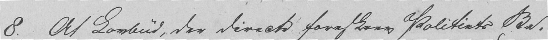8. At Lovbud, der directe foreskrev Politiets Be-
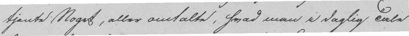tjente Noget, eller omtalte, hvad man i daglig Tale
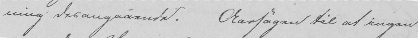ning desangaaende. Aarsagen til at ingen
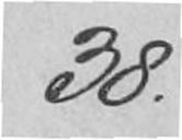38.
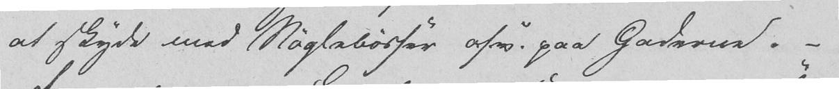at skyde med Nøglebøsser osv. paa Gaderne. -
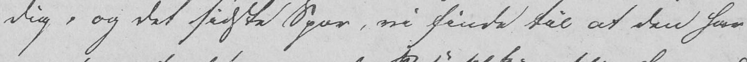dig, og det sidste Spor, vi finde til at den har
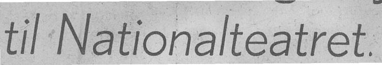til Nationalteatret.
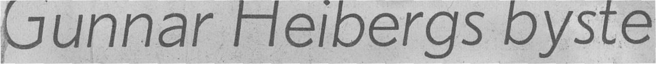Gunnar Heibergs byste
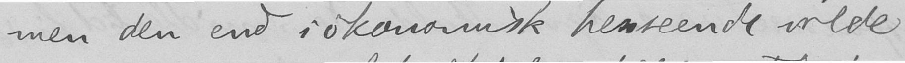men den end i økonomisk henseende vilde
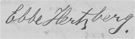Ebbe Hertzberg.
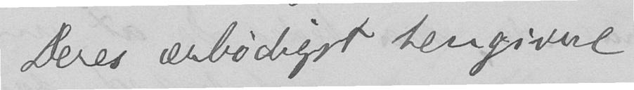Deres ærbødigst hengivne
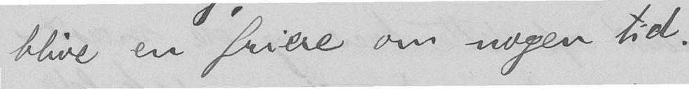blive en friere om nogen tid.
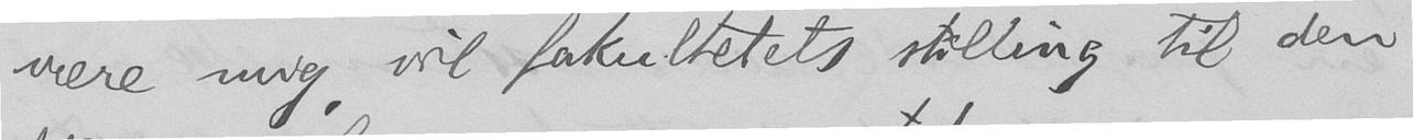være mig, vil fakultetets stilling til den
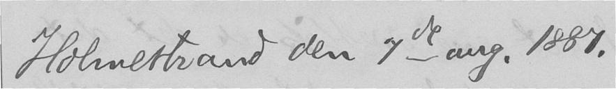Holmestrand den 7de aug. 1887.
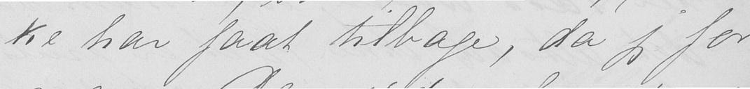ke har faat tilbage, da jeg for
